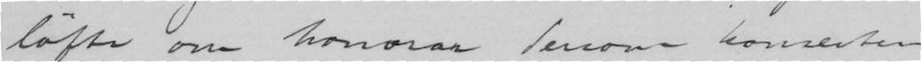løfte om honorar dersom konserten
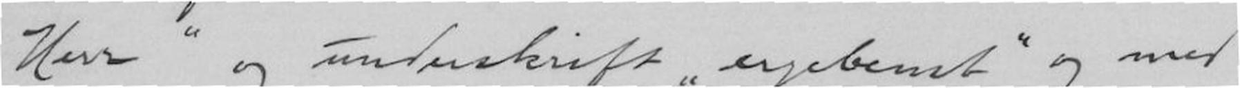Herr" og underskrift "ergebenst" og med
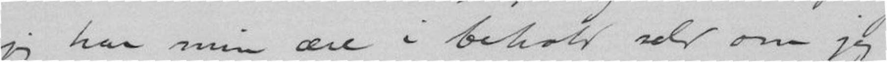jeg har min ære i behold selv om jeg
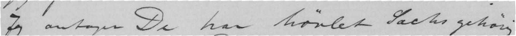Jeg antager De har høvlet Sachs gehørig,
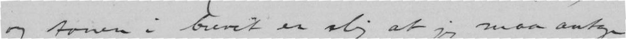og tonen i brevet er slig at jeg maa antage
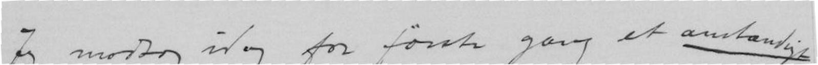Jeg modtog idag for første gang et anstændigt
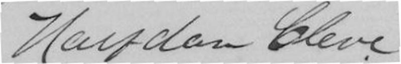Halfdan Cleve.
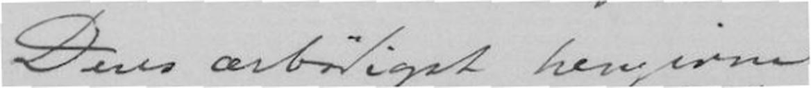Deres ærbødigst hengivne
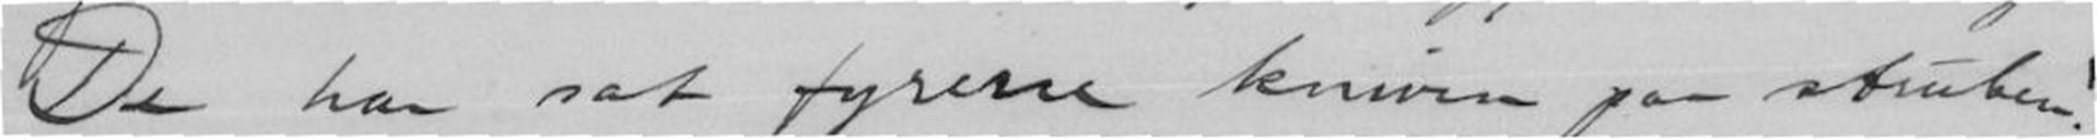De har sat fyrene kniven paa struben! "
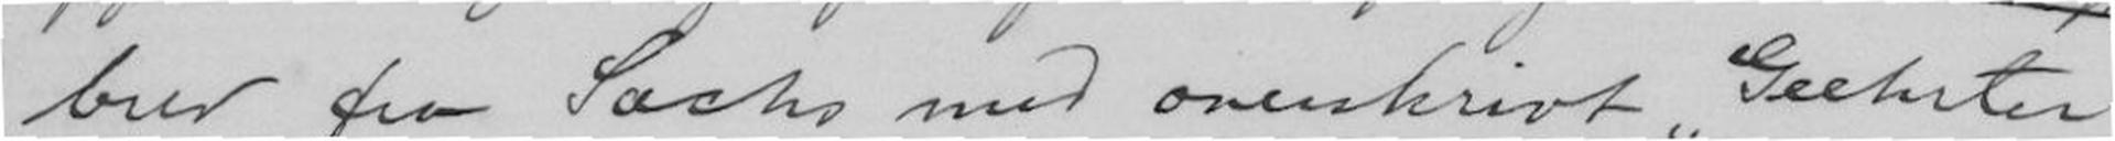brev fra Sachs med overskrivt "Geehrter
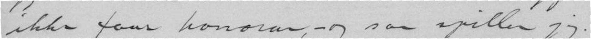ikke faar honorar, - og saa spiller jeg!
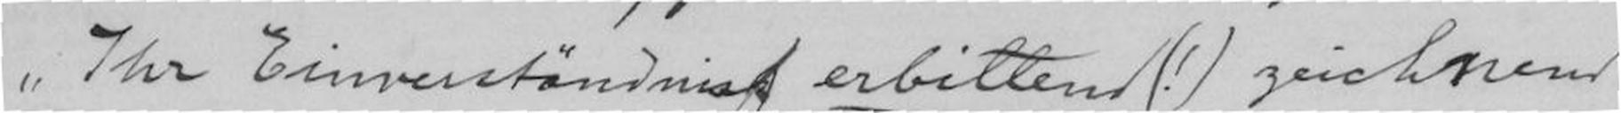Ihr Einverständnis erbittend(!) zeichnend
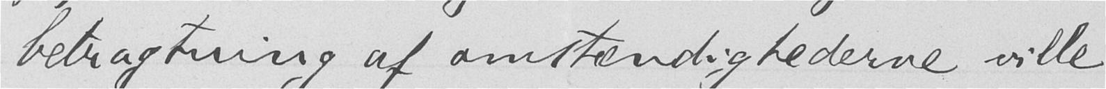betragtning af omstændighederne ville
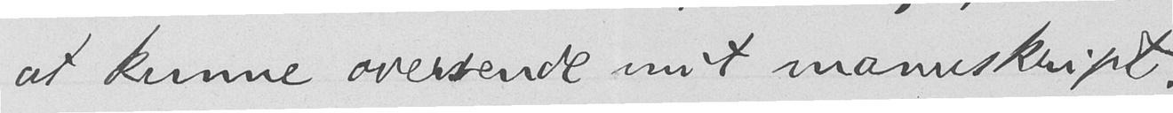at kunne oversende mit manuskript.
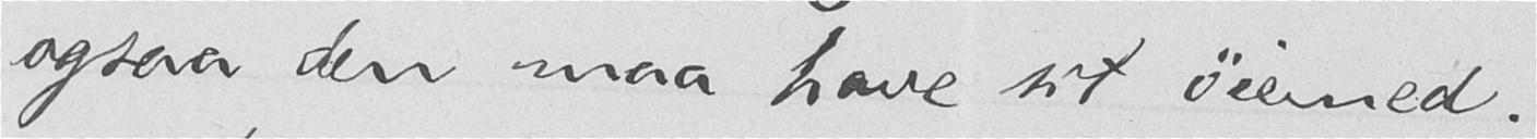ogsaa den maa have sit øiemed.
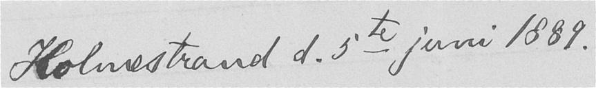Holmestrand d. 5te juni 1889.
